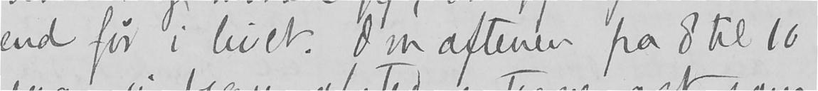end før i livet. Om aftenen fra 8 til 10
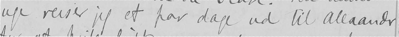uge reiser jeg et par dage ud til Alexander
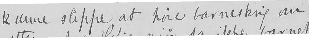kunne slippe at høre barneskrig om
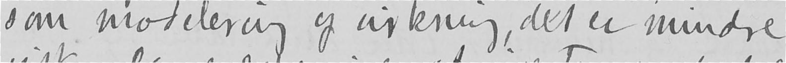som modelering og viskning, det er mindre
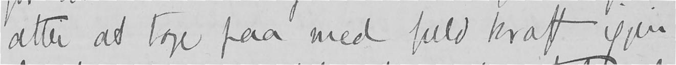atter at tage paa med fuld kraft igjen.
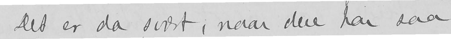Det er da svært, naar dere har saa
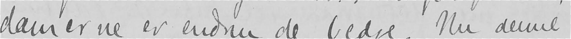damerne er endnu de bedre. Nu denne
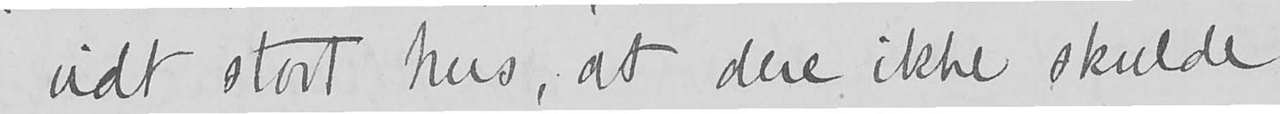vidt stort hus, at dere ikke skulde
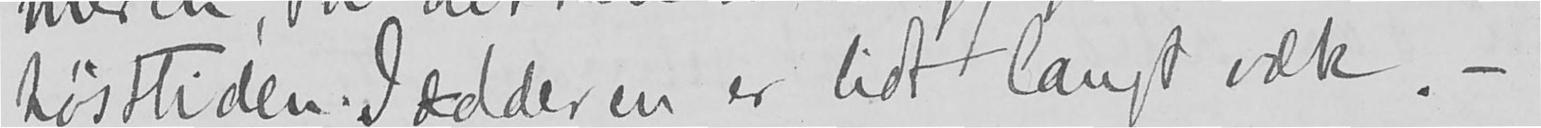høsttiden. Jædderen er lidt langt væk. -
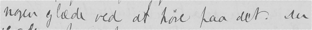nogen glæde ved at høre paa det. Du
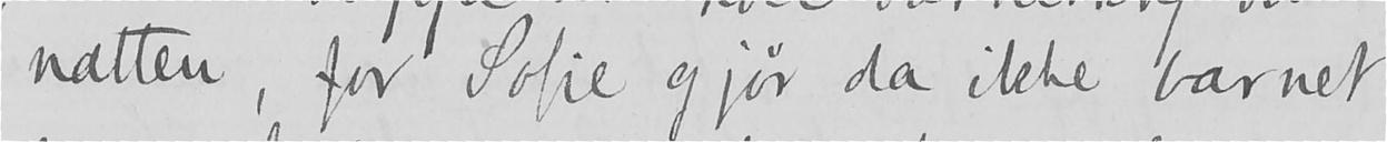natten, for Sofie gjør da ikke barnet
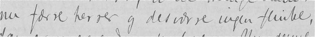nu færre herrer og desværre ingen flinke,
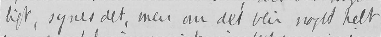ligt, synes det, men om det blir noget helt
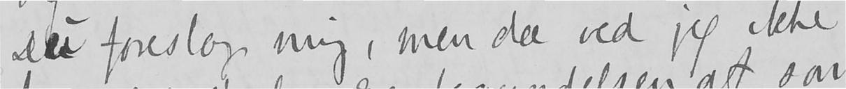Du foreslog mig, men da ved jeg ikke
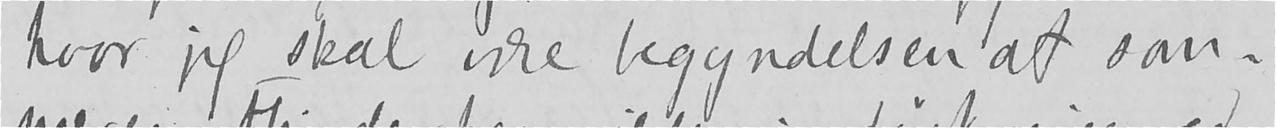hvor jeg skal være begyndelsen af som-
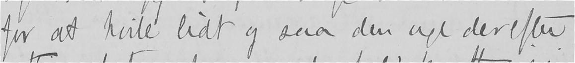for at hvile lidt og saa den uge derefter
